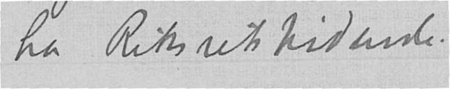ha Riksretstidende.
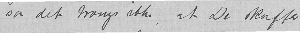saa det trængs ikke, at De skaffer
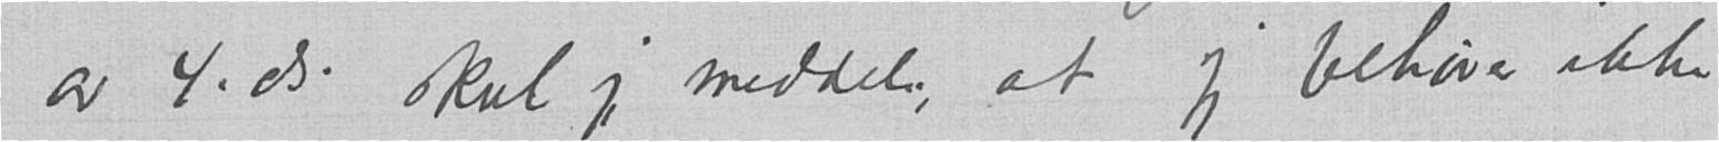av 4. ds. skal jeg meddele, at jeg behøver ikke
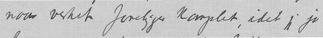naar verket foreligger komplet, idet jeg jo
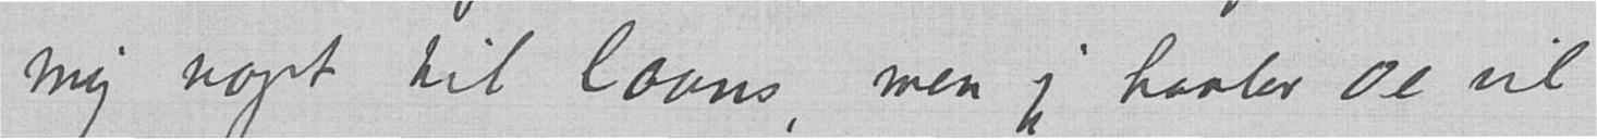mig noget til laans, men jeg haaber De vil
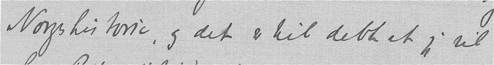Norgeshistorie, og det er til dette at jeg vil
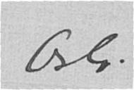Oslo.
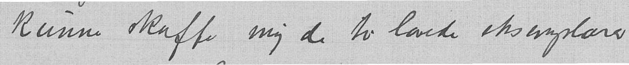kunne skaffe mig de to lovede eksemplarer
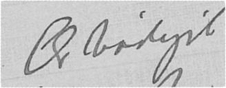Ærbødigst
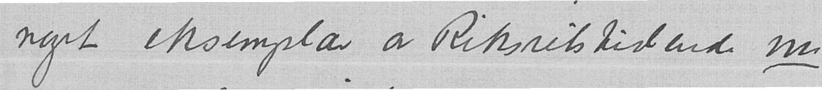noget eksemplar av Riksretstidende nu,
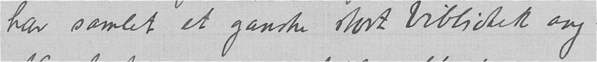har samlet et ganske stort bibliotek ang.
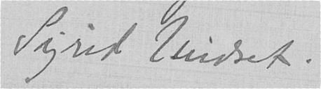Sigrid Undset.
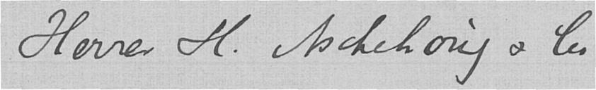Herrer H. Aschehoug & Co
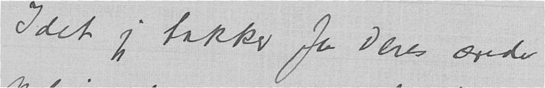Idet jeg takker for Deres ordre
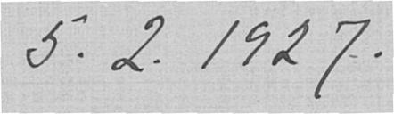5.2.1927.
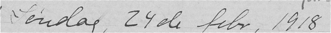Søndag, 24de febr, 1918
